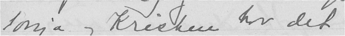Sonja og Kristen har det
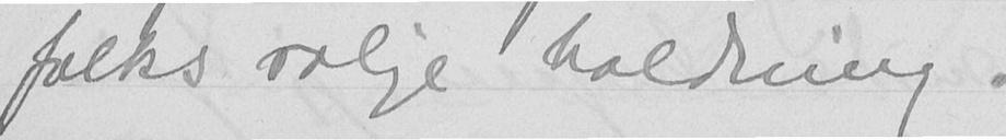folks rolige holdning.
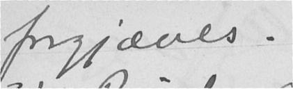forgjæves.
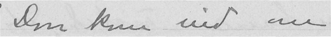Don kom ind om
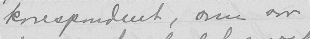korespondent, som var
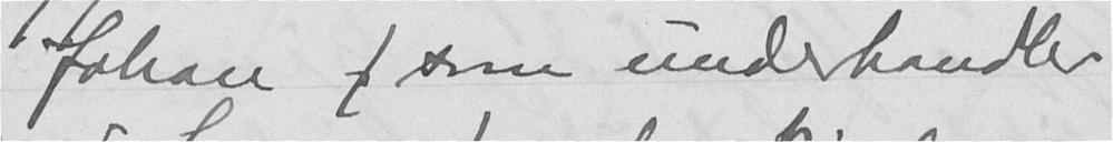Johan (som underhandler
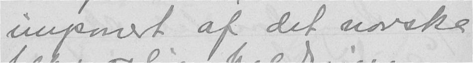imponert af det norske
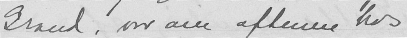Grand, var om aftenen hos
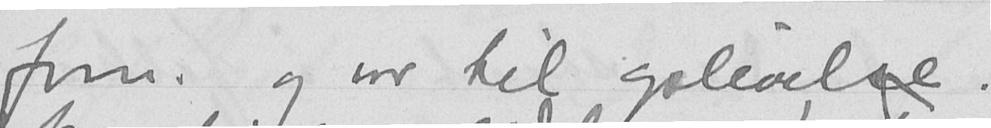form. og var til oplivelse.
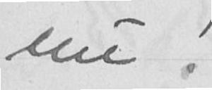nu!
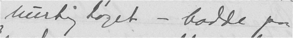hurtigtoget - bodde paa
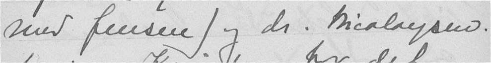med Jensen) og dr. Nicolaysen.
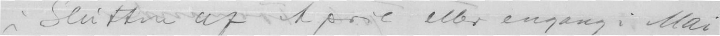i Slutten af April eller engang i Mai
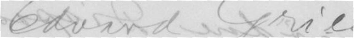Edvard Grieg
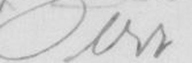Herr
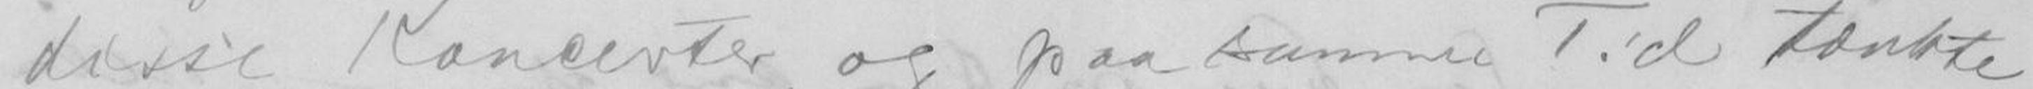disse Koncerter, og paa samme Tid tænkte
